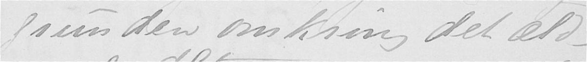grunden omkring det æld-
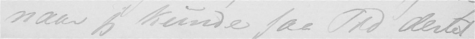naar jeg kunde faa Tid dertil
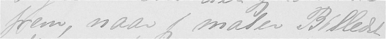frem, naar jeg maler Billedet.
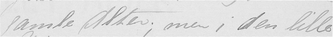gamle Alter; men i den lille
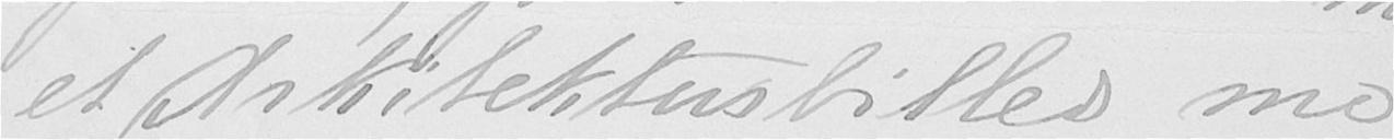et Arkitekturbillede med
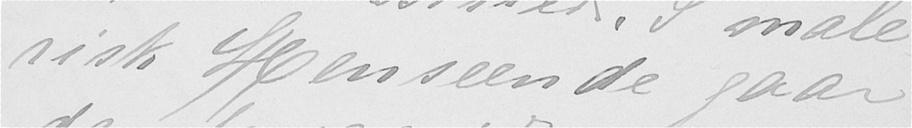risk Henseende gaar
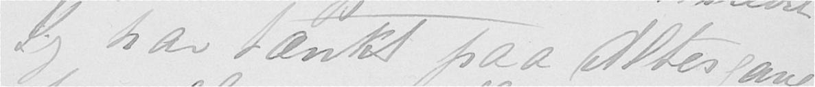Jeg har tænke paa Altergang-
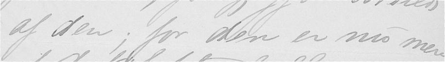af den, for den er nu mer
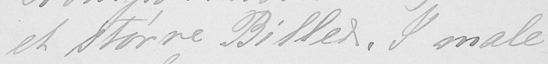et større Billede. I male-
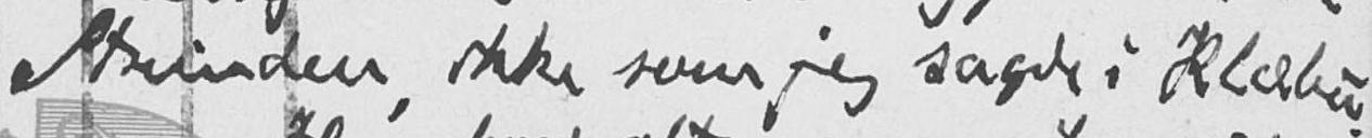Strinden, ikke som jeg sagde i Klæbu.
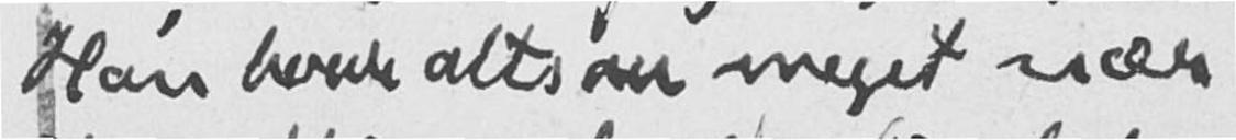Han boede altsaa meget nær
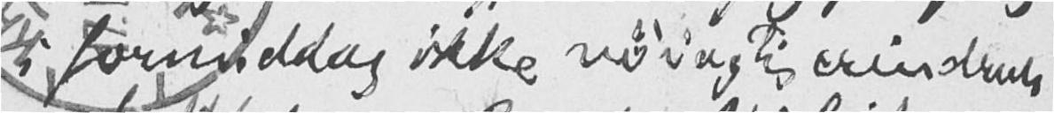i formiddag ikke nøiagtig erindrede
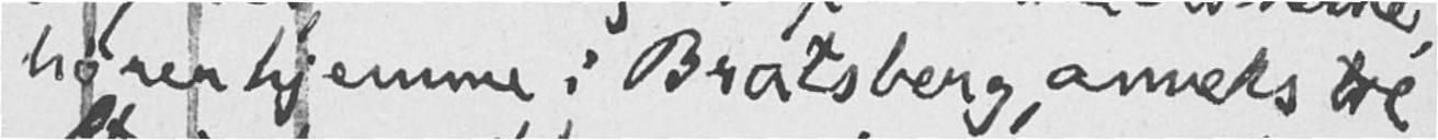hører hjemme i Bratsberg, omreks til
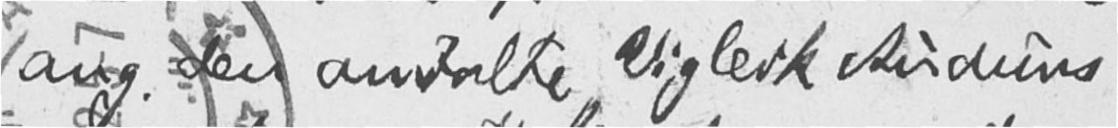ang. den omtalte Vigleik Auduns-
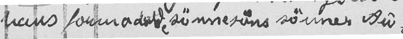hans fonnadald, sønnesøns sønner Au-
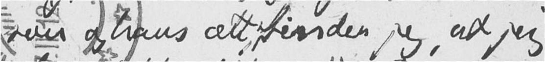son og hans ætt, finder jeg, at jeg
